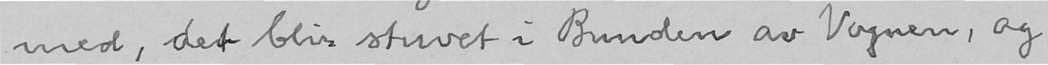med, det blir stuvet i Bunden av Vognen, og
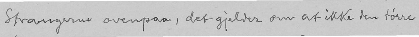Strangerne ovenpaa, det gjelder om at ikke den tørre
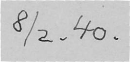8/2. 40.
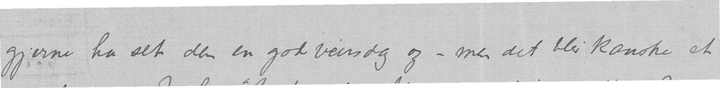gjerne ha set den en godveirsdag og – men det blir kanske et
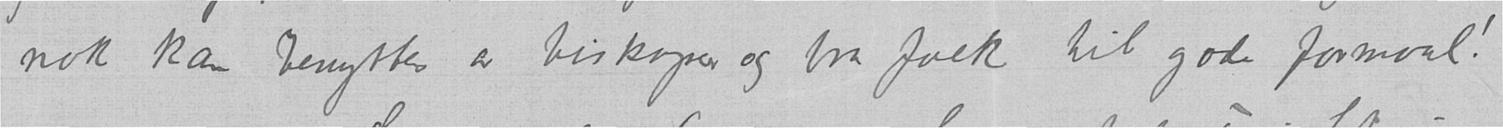nok kan benyttes av biskoper og bra folk til gode formaal!
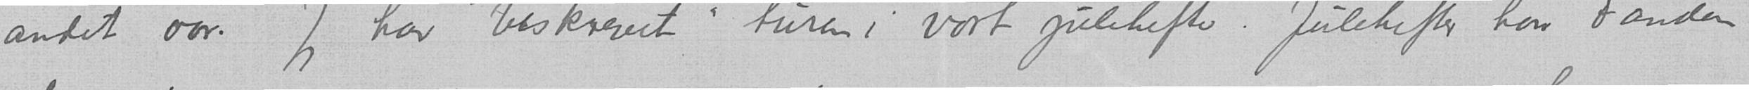andet aar. Jeg har "beskrevet" turen i vort julehefte. Julehefter har Fanden
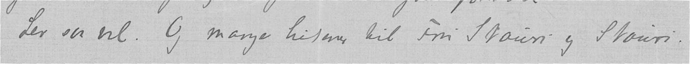Lev saa vel. Og mange hilsener til Fru Stauri og Stauri.
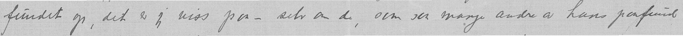fundet op, det er jeg viss paa – selv om de, som saa mange andre av hans paafund
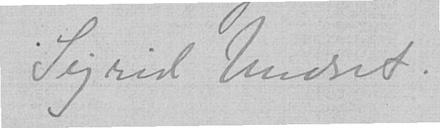Sigrid Undset.
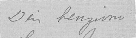Din hengivne
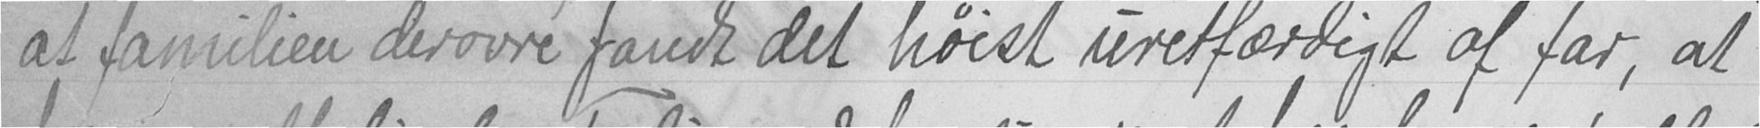at familien derovre fandt det høist uretfærdigt af far, at
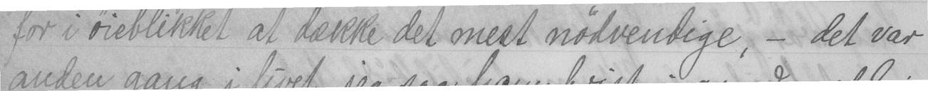for i øieblikket at dække det mest nødvendige, - det var
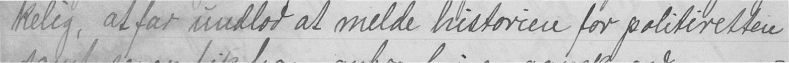kelig, at far undlod at melde historien for politiretten
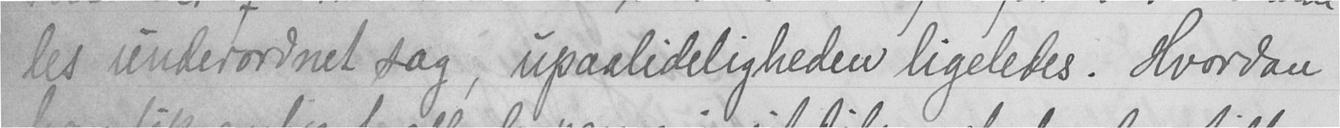les underordnet sag, upaalideligheden ligeledes. Hvordan
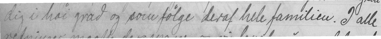dig i høi grad og som følge deraf hele familien. I alle
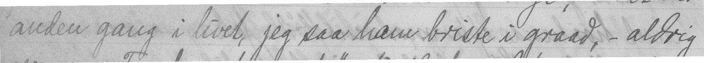anden gang i livet, jeg saa ham briste i graad, - aldrig
